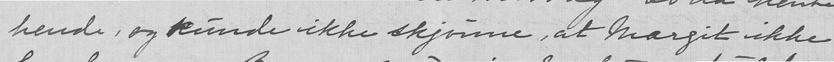hende, og kunde ikke skjønne, at Margit ikke
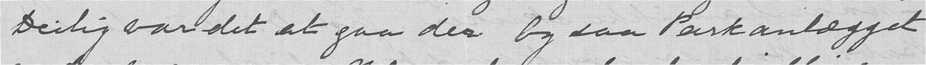Deilig var det at gaa der. Og saa Parkanlægget
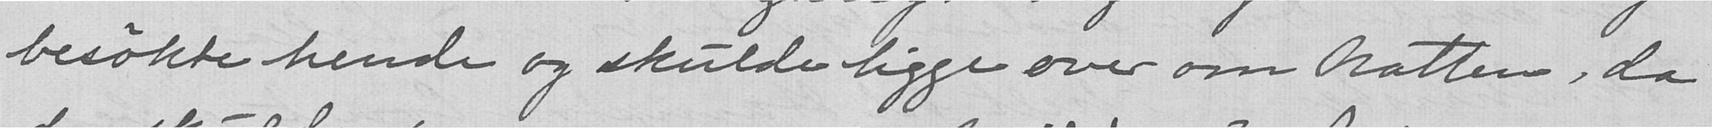besøkte hende og skulde ligge over om Natten, da
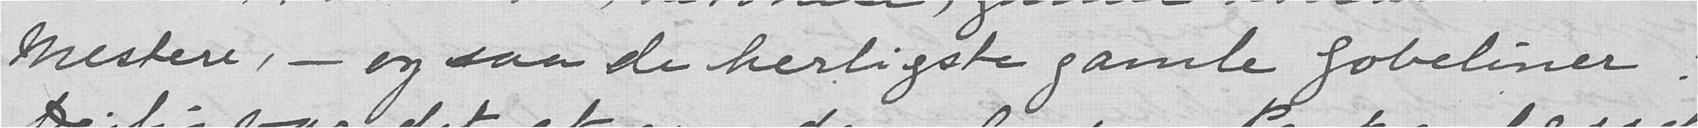mestere, - og saa de herligste gamle gobeliner!
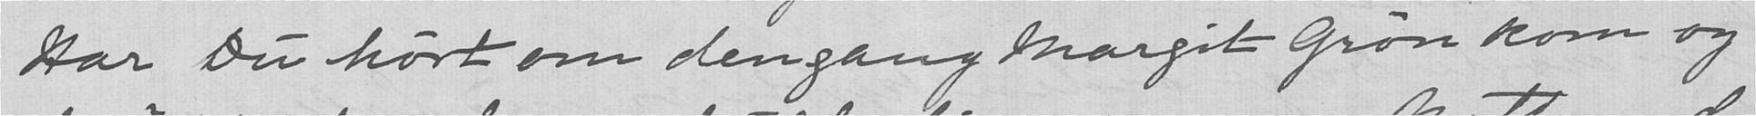Har Du hørt om dengang Margit Grøn kom og
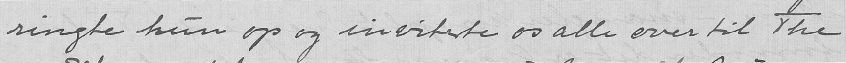ringte hun op og inviterte os alle over til The
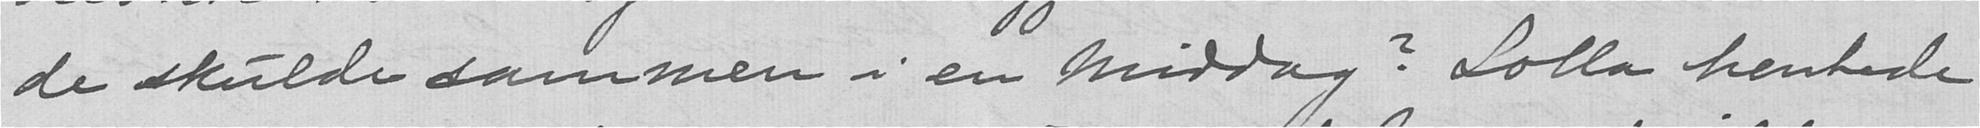de skulde sammen i en Middag? Lolla hentede
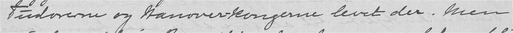Tudorerne og Hanover-kongerne levet der. Men
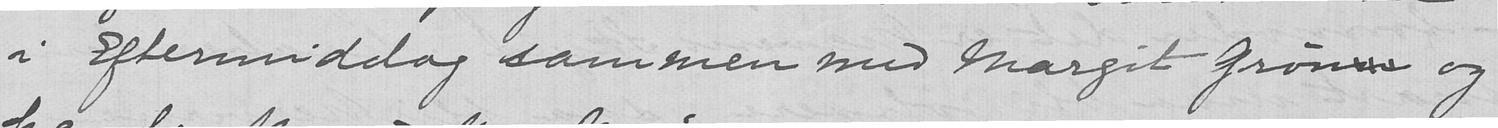i Eftermiddag sammen med Margit Grøn og
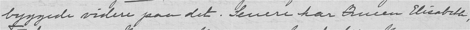byggede videre paa det. Senere har Queen Elisabeth,
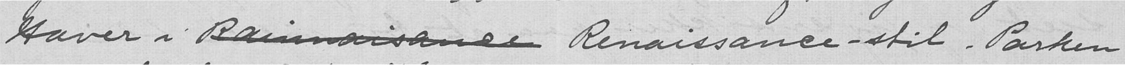Haver i  Renaissance-stil. Parken
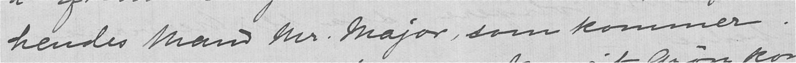hendes Mand Mr. Major, som kommer.
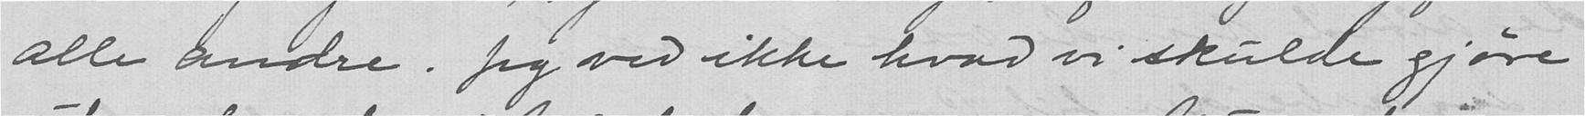alle andre. Jeg ved ikke hvad vi skulde gjøre
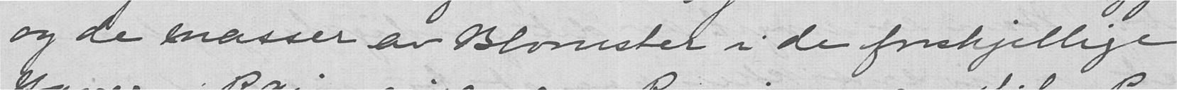og de masser av Blomster i de forskjellige
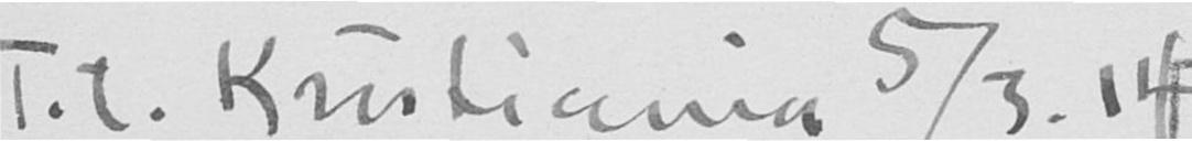T.t. Kristiania 5/3.14
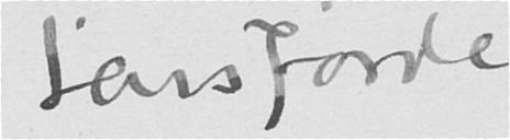Lars Jorde
